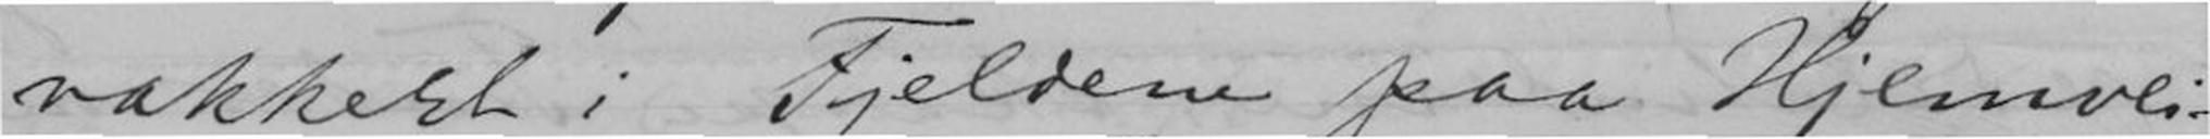vakkert i Fjeldene paa Hjemvei-
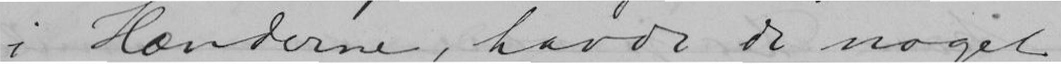i Hænderne, havde de noget
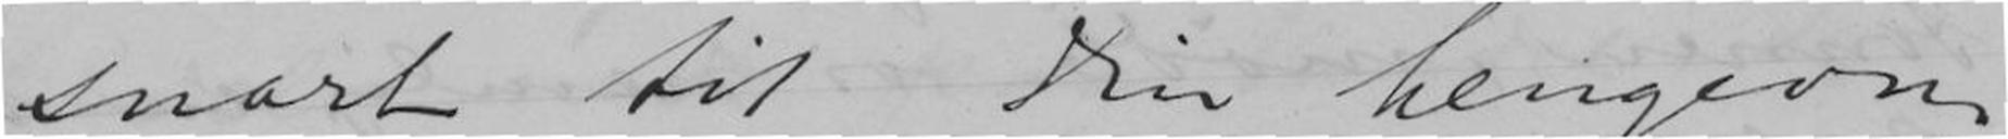snart til Din hengivne
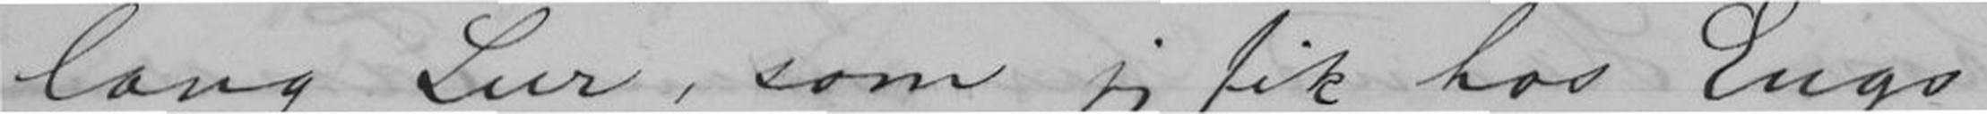lang Lur, som jeg fik hos Engs
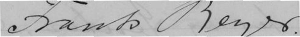Frants Beyer
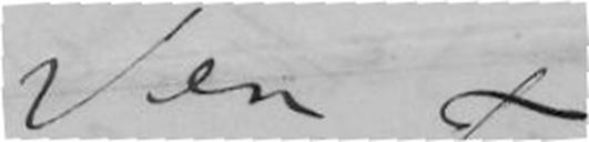Ven
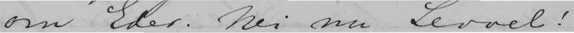om Eder. Nei nu Levvel!
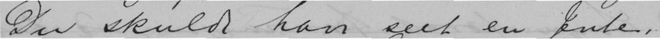Du skulde have seet en Jente,
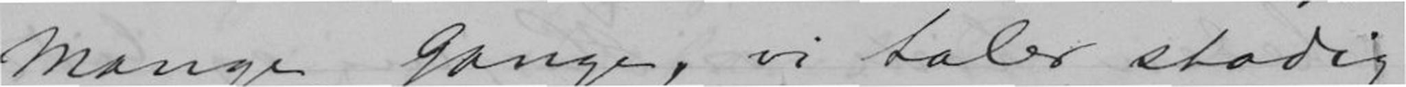Mange Gange, vi taler stadig
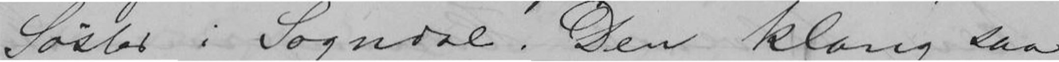Søster i Sogndal. Den klang saa
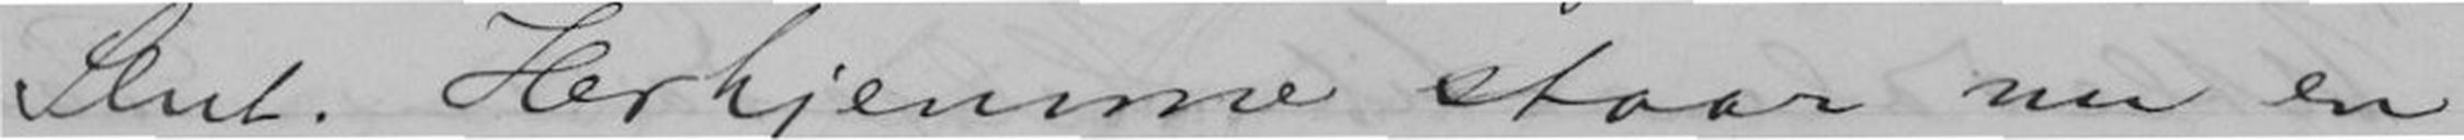Slut. Her hjemme staar nu en
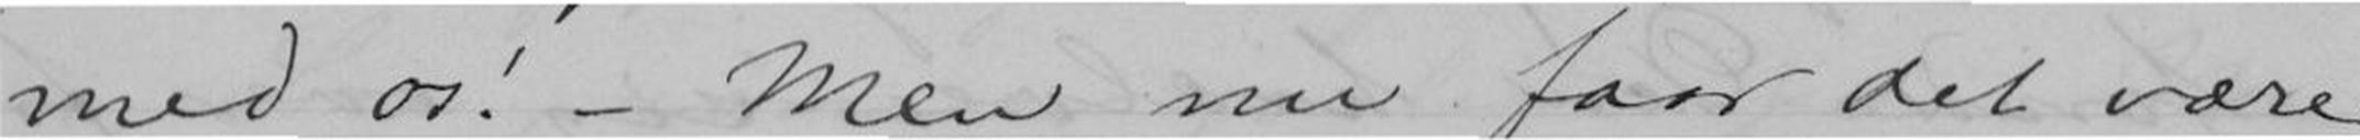med os! - Men nu faar det være
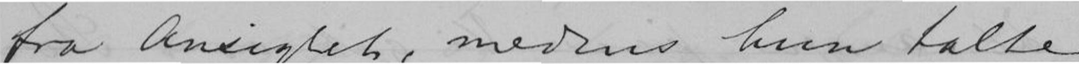fra Ansigtet, medens hun talte
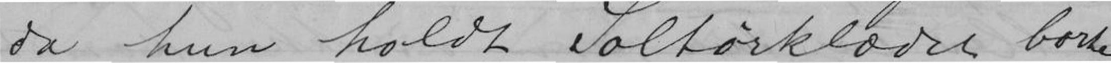da hun holdt Soltørklædet borte
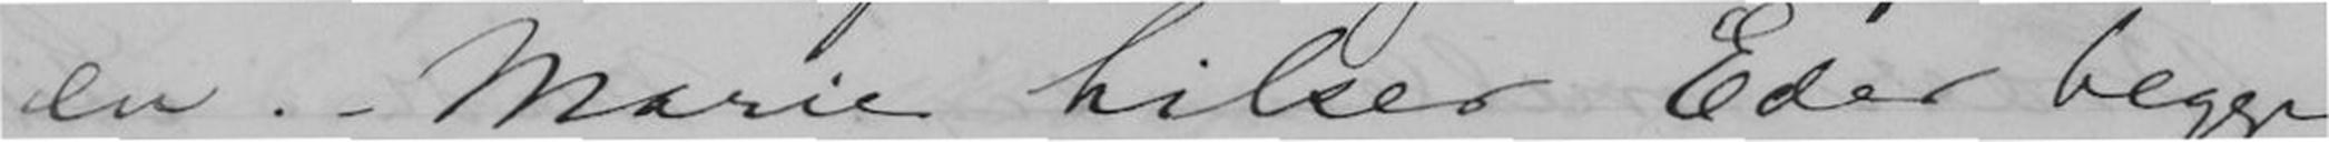en. - Marie hilser Eder begge
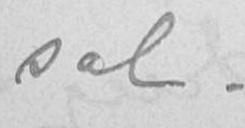sal.
